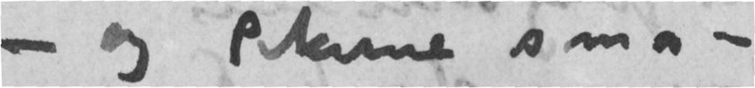– og Pikene små –
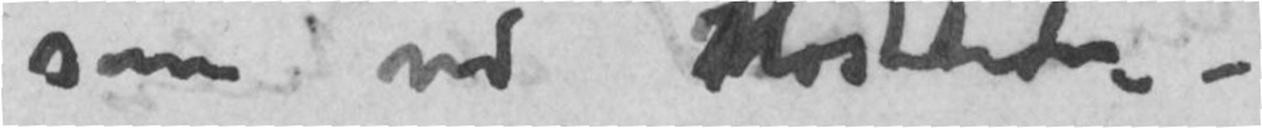som ved Høsttiden –
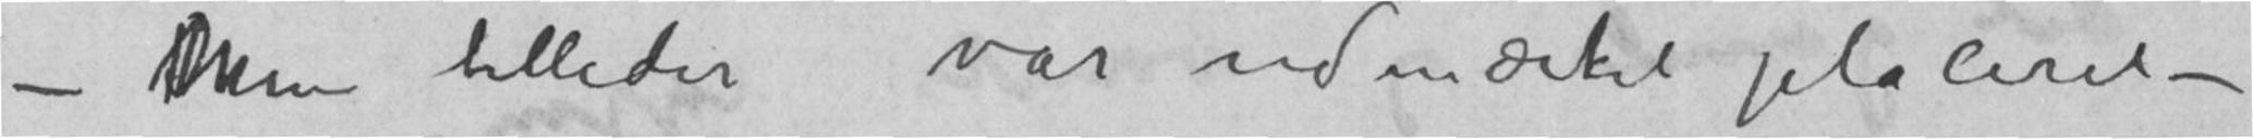– Mine billeder var udmærket placeret –
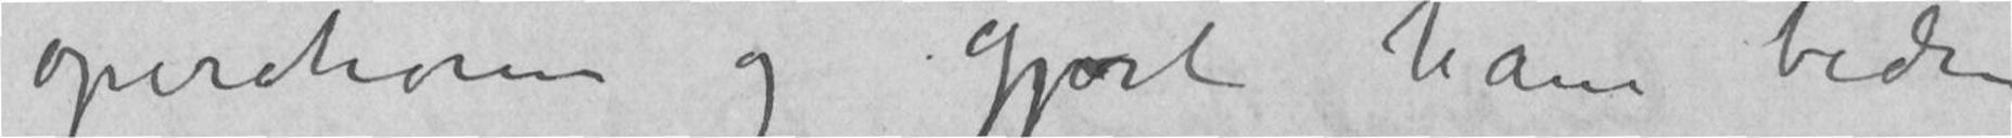operationen og gjort ham bedre
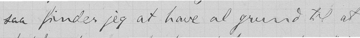saa finder jeg at have al grund til at
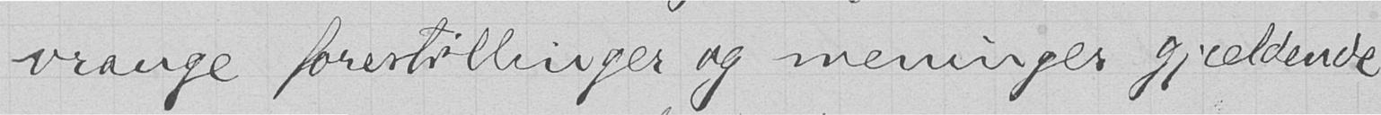vrange forestillinger og meninger gjældende
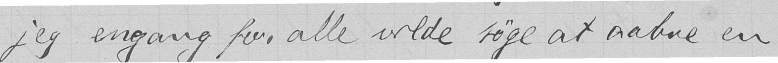jeg engang for alle vilde søge at aabne en
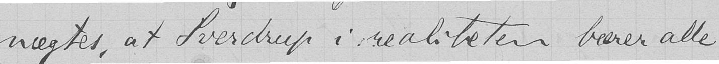nægtes, at Sverdrup i realiteten bærer alle
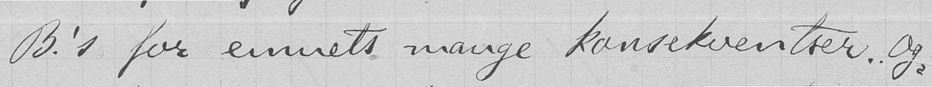B.’s for emnets mange konsekventser. Og-
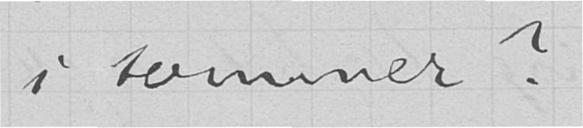i sommer?
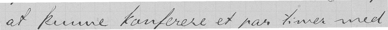at kunne konferere et par timer med
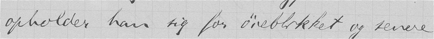opholder han sig for øieblikket og senere
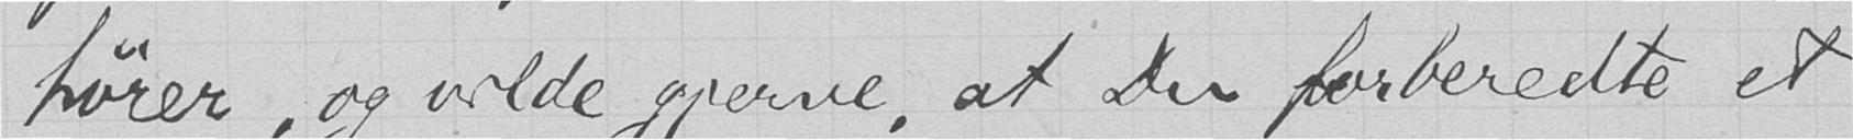hører, og vilde gjerne, at Du forberedte et
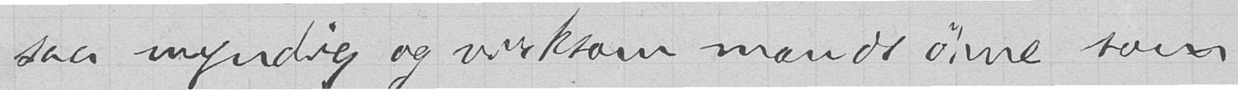saa myndig og virksom mands øine som
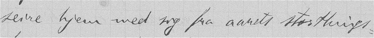seire hjem med sig fra aarets storthings-
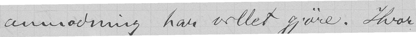anmodning har villet gjøre. Hvor
